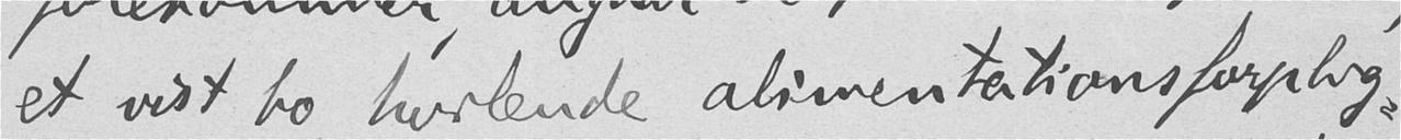et vist bo, hvilende alimentationsforplig-
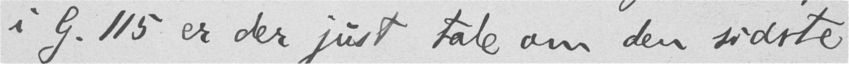i G. 115 er der just tale om den sidste
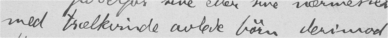med trælkvinde avlede børn, derimod
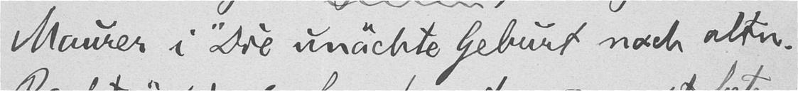Maurer i "Die unachte Geburt noch altn.
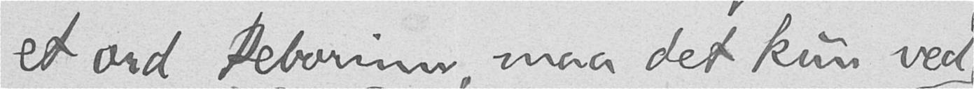et ord þeborinn, maa det kun ved
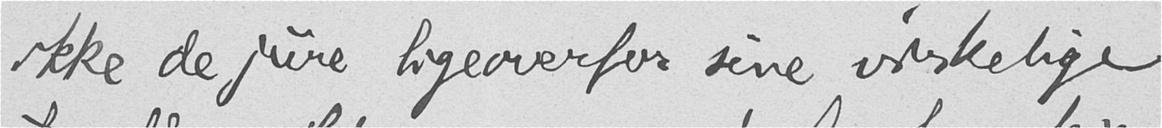ikke de jure ligeoverfor sine virkelige
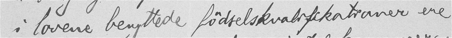i lovene benyttede fødselskvalifikationer ere
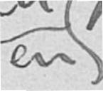en
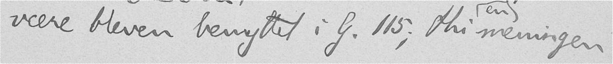være bleven benyttet i G. 115; thi meningen
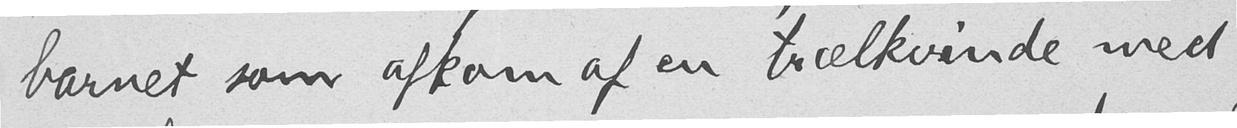barnet som afkom af en trælkvinde med
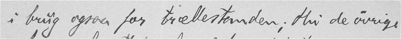i brug ogsaa for trællestanden; thi de øvrige
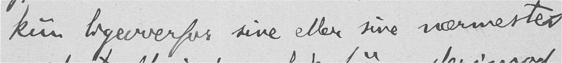kun ligeoverfor sine eller sine nærmestes
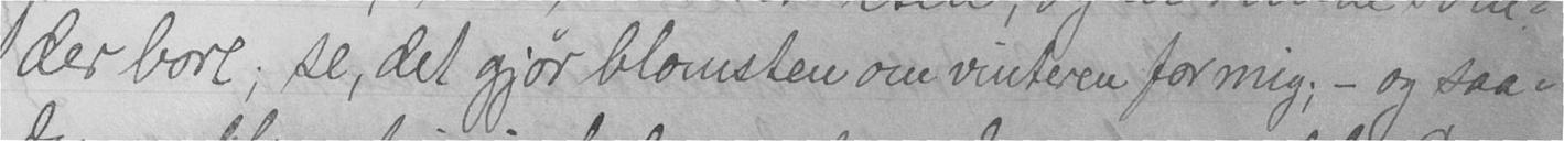der bort; se, det gjør blomsten om vinteren for mig; - og saa-
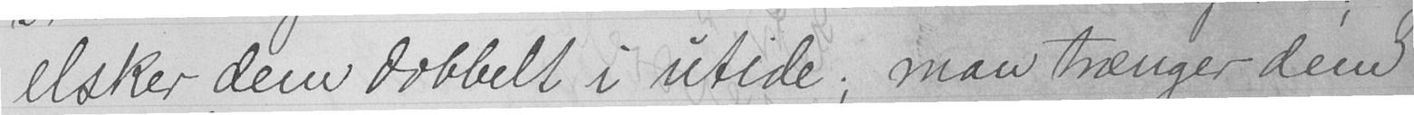elsker dem dobbelt i utide; man trenger dem
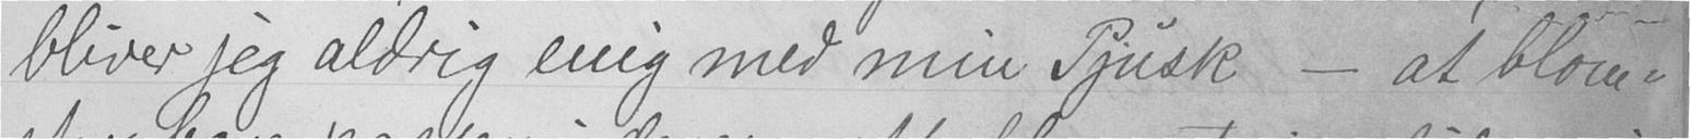bliver jeg aldrig enig med min Pjusk - at blom-
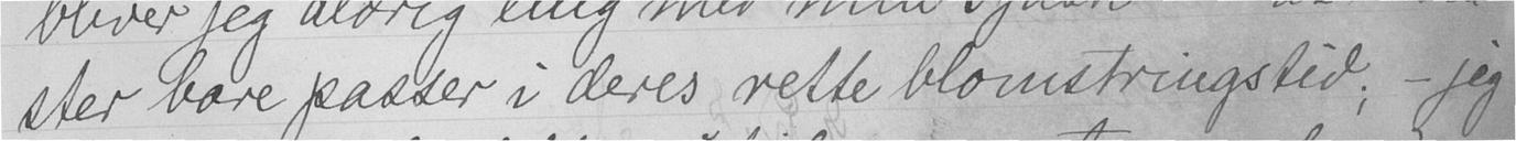ster bare passer i deres rette blomstringstid; - jeg
